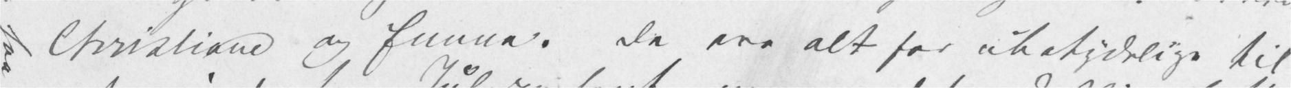Christiane og Emma. De ere alt for ubetydelige til
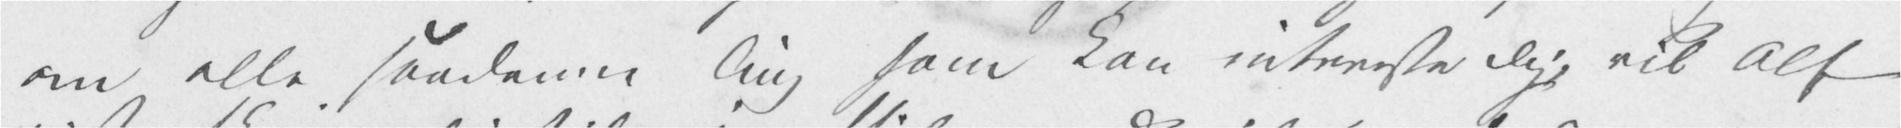om alle saadanne Ting som kan interessere Dig, vil Alf
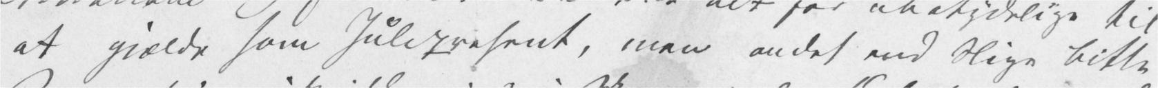at gjælde som Julepresent, men andet end Slige bitte
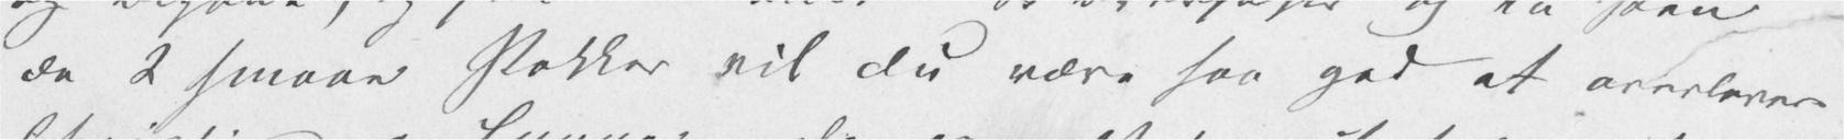de 2 smaae Pakker vil Du være saa god at overlevere
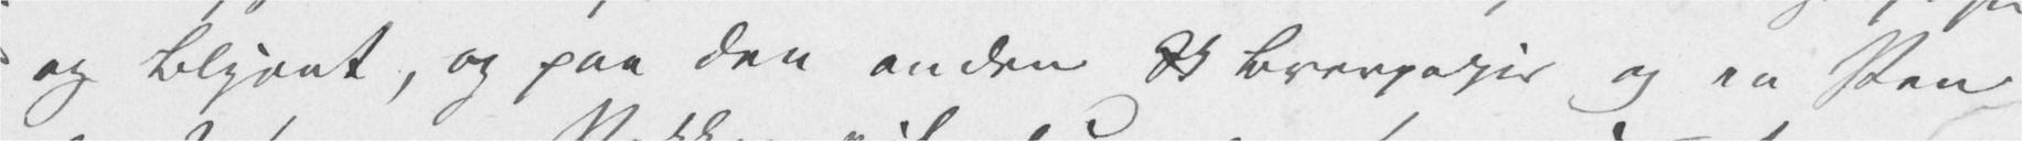og Blyant, og paa den anden Sk Brevpapir og en Pen
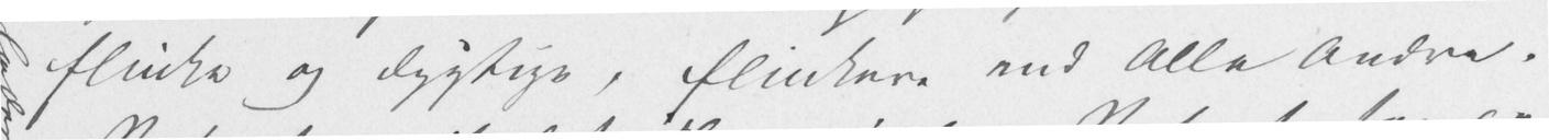flinke og dygtige, flinkere end Alle Andre.
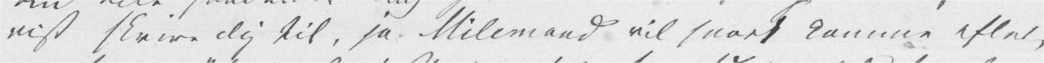vist skrive Dig til, ja Milemand vil snart komme efter,
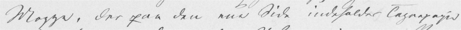Mappe, der paa den ene Side indeholder Tegnepapir
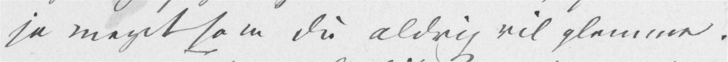ja meget som Du aldrig vil glemme.
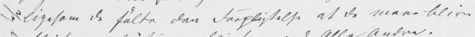ligesom de følte den Forpligtelse at de maae blive
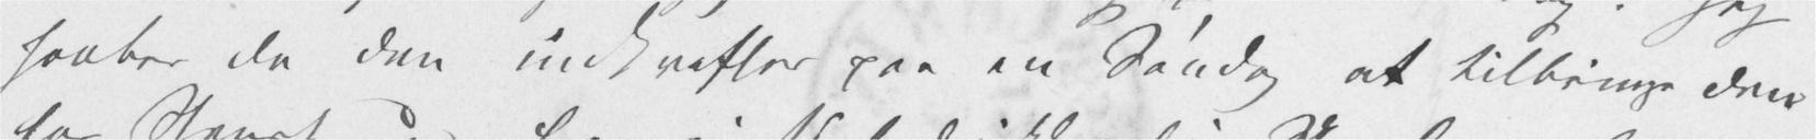haaber da den indtreffer paa en Søndag at tilbringe den
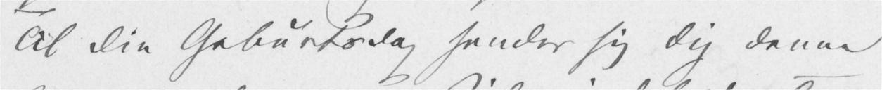Til Din Geburtsdag sender jeg Dig denne
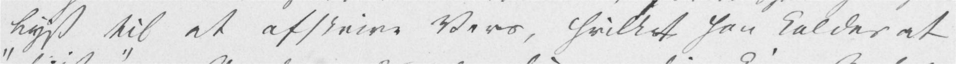Lyst til at afskrive Vers, hvilket han kalder at
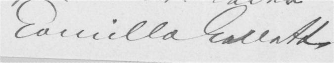Camilla Collett
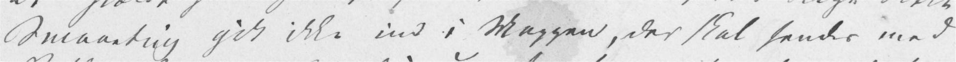Smaaeting gikk ikke ind i Mappen, der skal sendes med
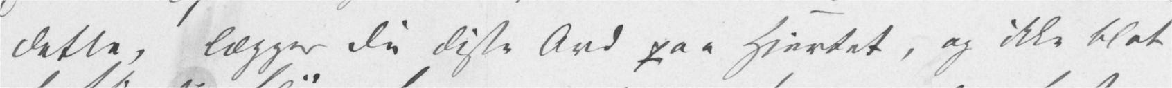dette, lægger Du disse Ord paa Hjertet, og ikke blot
